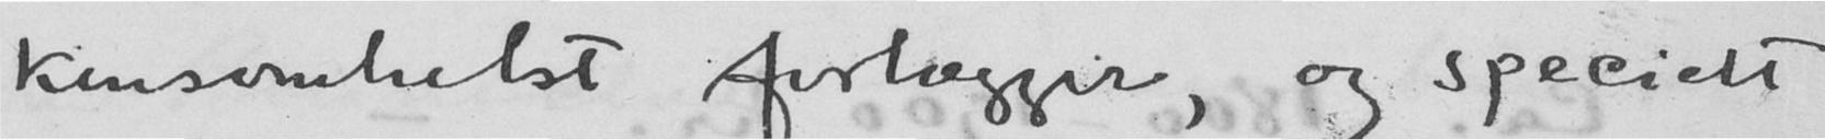kensomhelst forlægger, og specielt
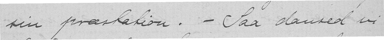sin præstation. - Saa dansed vi
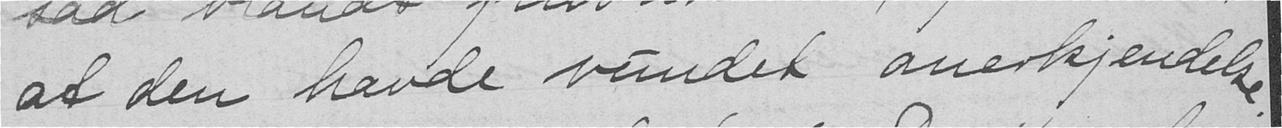at den havde vundet anerkjendelse.
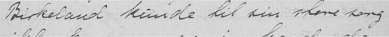Birkeland kunde til sin store sorg
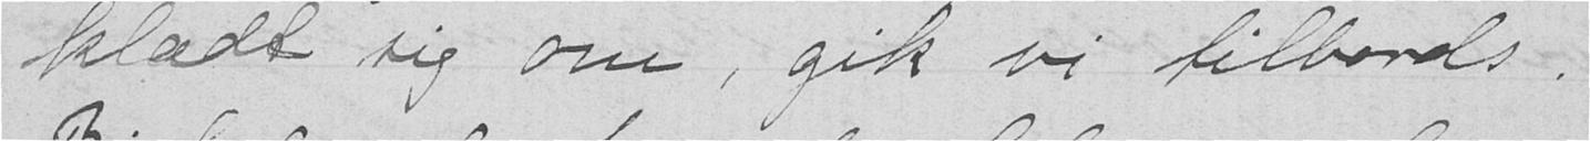klædt sig om, gik vi tilbords.
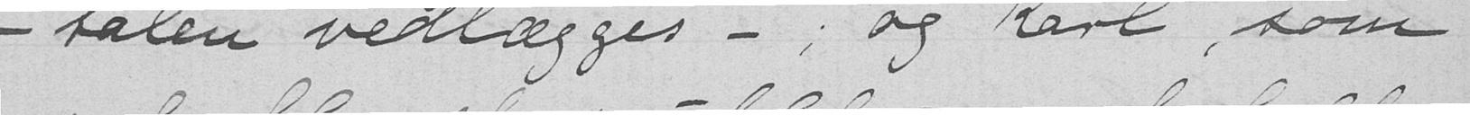- talen vedlægges - ; og Karl, som
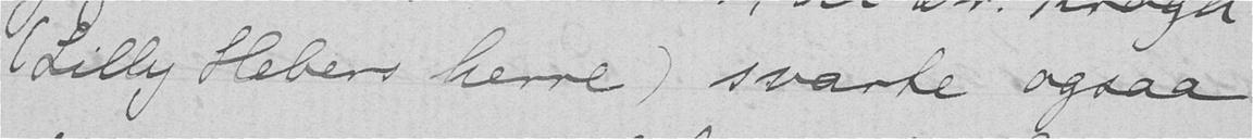(Lilly Hebers herre) svarte ogsaa
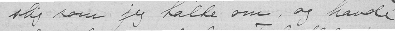slig som jeg talte om, og havde
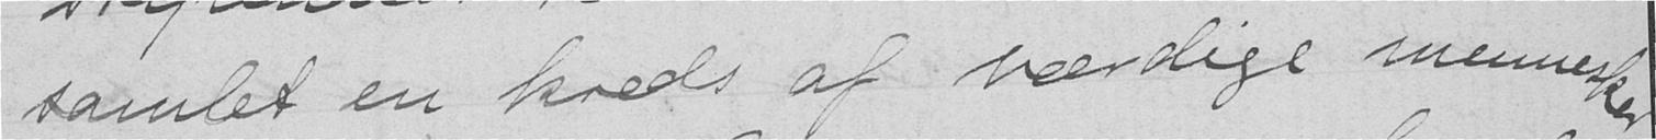samlet en kreds af værdige mennesker
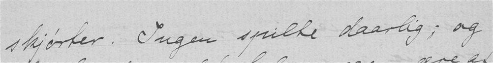skjørter. Ingen spilte daarlig; og
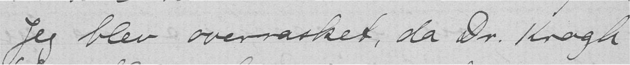Jeg blev overrasket, da Dr. Krogh
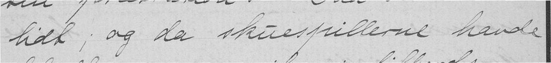lidt; og da skuespillerne havde
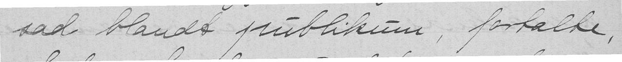sad blandt publikum, fortalte,
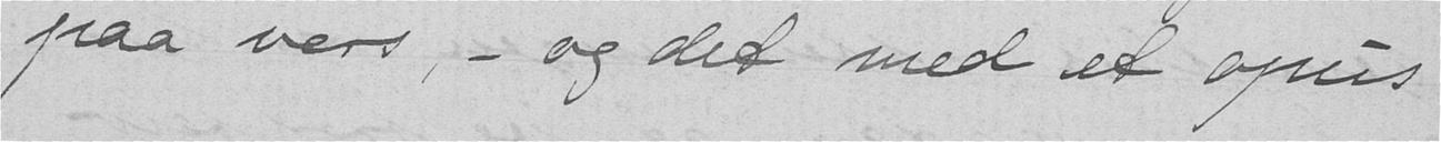paa vers, - og det med et opus
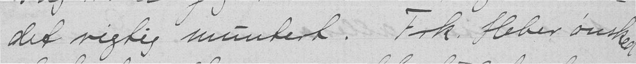det rigtig muntert. Frk. Heber ønsked
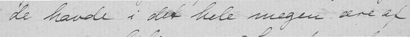de havde i det hele megen ære af
